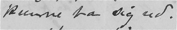kunne ta sig ud.
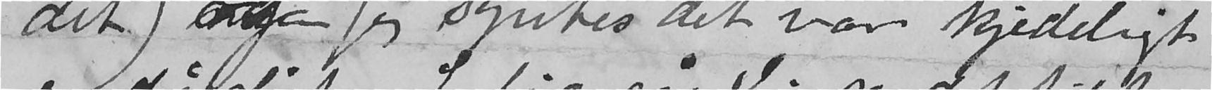det) og jeg syntes det var kjedeligt
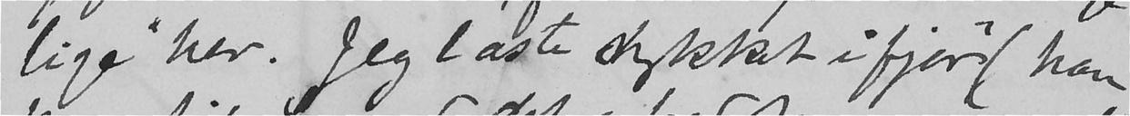lige her. Jeg læste stykket ifjor (han
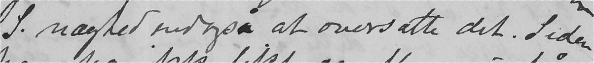S. nægted endogså at oversætte det. Siden
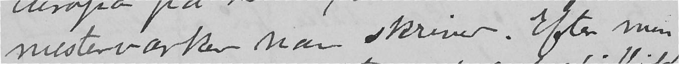mesterværker han skriver. Efter min
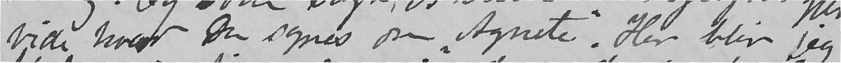vide hvad Du synes om "Agnete". Her blir jeg
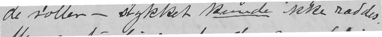de roller - stykket kunde ikke reddes.
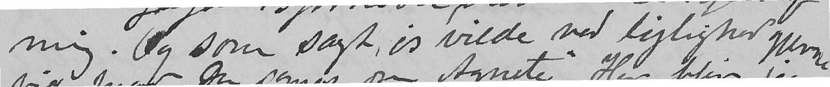mig. Og som sagt, jeg vilde ved lejlighed gjerne
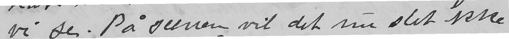vi se. På scenen vil det nu slet ikke
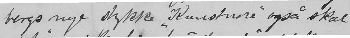bergs nye stykke "Kunstnere" også skal
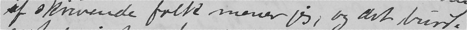af skrivende folk mener jeg, og det burde
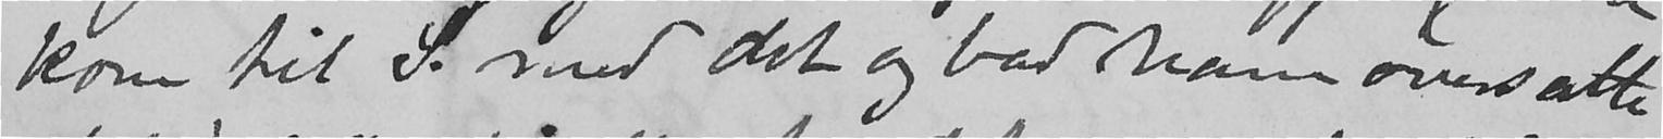kom til  med det og bad ham oversætte
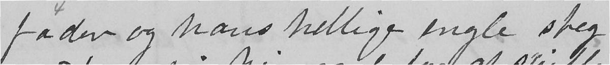fader og hans hellige engle steg
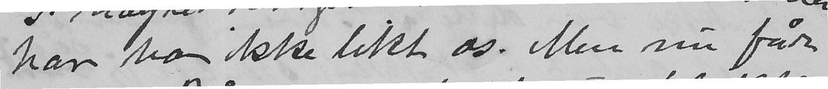har han ikke likt os. Men nu får
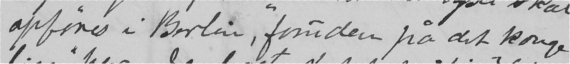opføres i Berlin, foruden fra det konge
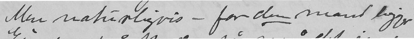Men naturligvis - for den mant ligger
